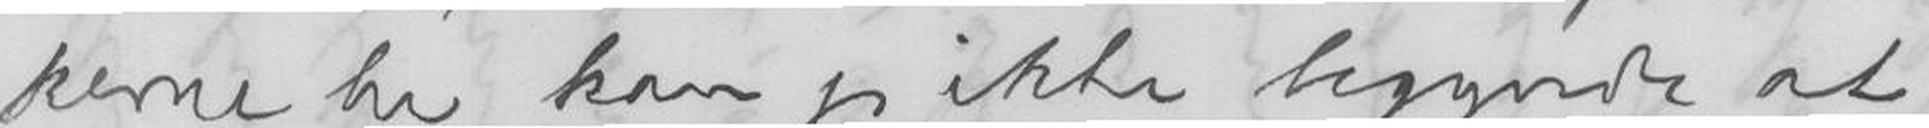kerne her kan jeg ikke begynde at
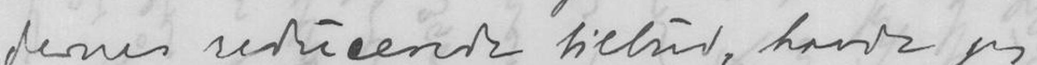dernes reducerede tilbud, havde jeg
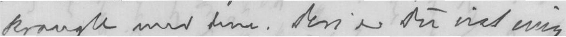krangle med dem. Deri er Du vist mig
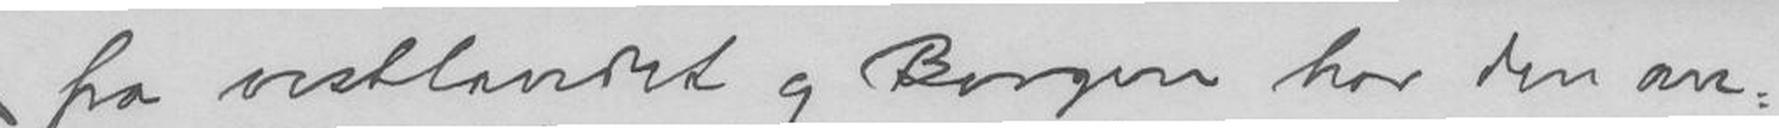fra vestlandet og Bergen har den an-
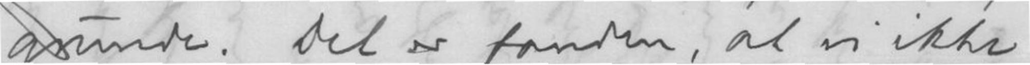grunde. Det er fanden, at vi ikke
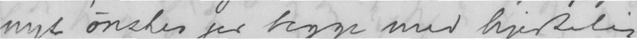nyt ønskes jer begge med hjertelig
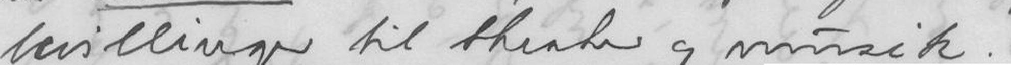bevillinger til theater og musik.
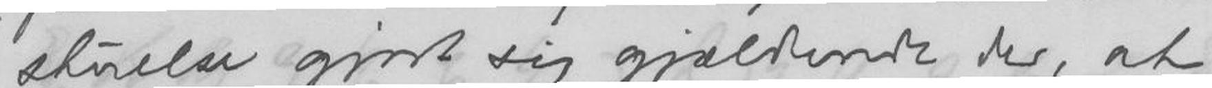skuelse gjort sig gjældende der, at
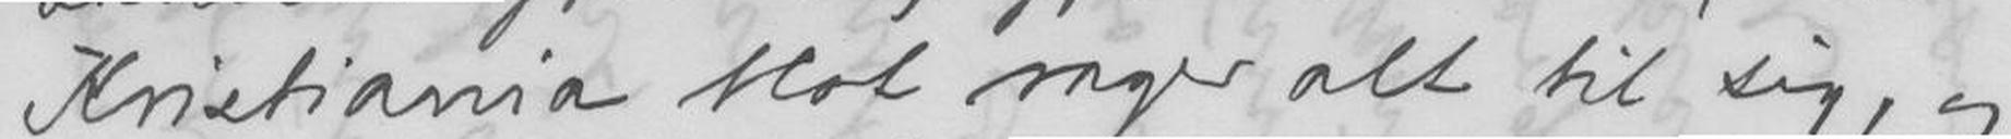Kristiania blot tager alt til sig, og
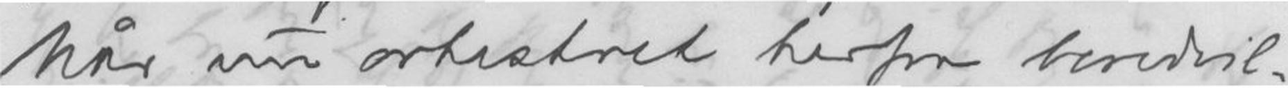Når nu orkestret herfra beredvil-
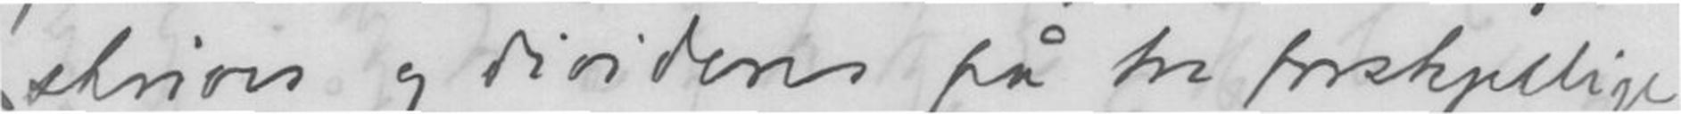skrives og divideres på tre forskjellige
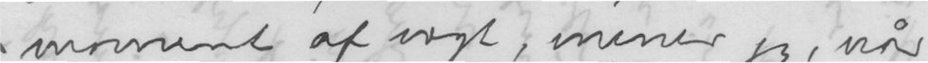moment af nogle, mener jeg, når
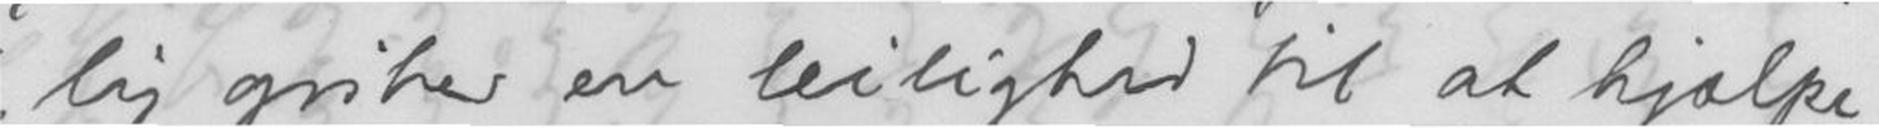lig griber en leilighed til at hjælpe
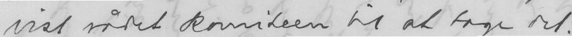vist rådet komiteen til at tage det.
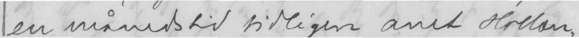en månedstid tidligere anet Hollæn-
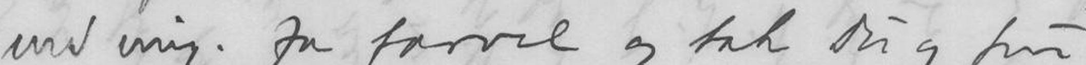med mig. Ja farvel og tak Du og fru
